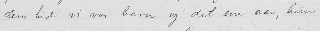den tid vi var barn og det ene aar, hun
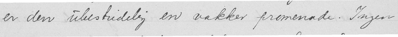er den ubestridelig en vakker promenade. Ingen
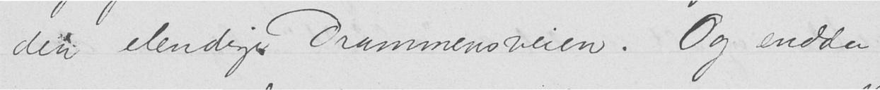den elendige Drammensveien. Og endda
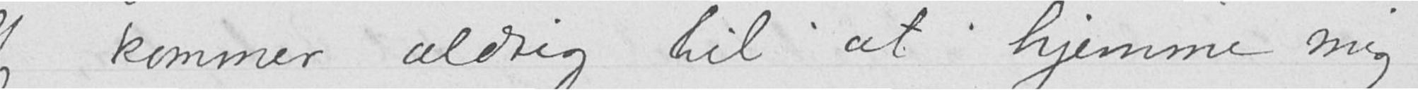Jeg kommer aldrig til at hjemme mig
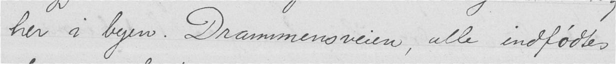her i byen. Drammensveien, alle indfødtes
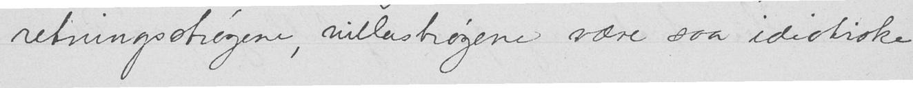retningsstrøgene, villastrøgene være saa idiotiske
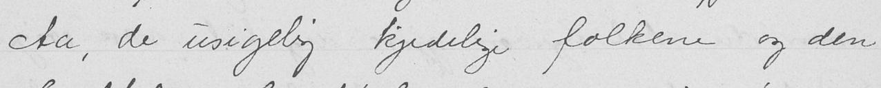Aa, de usigelig kjedelige folkene og den
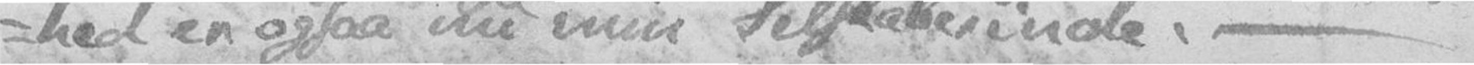-hed er ogsaa nu min Selskaberinde. –
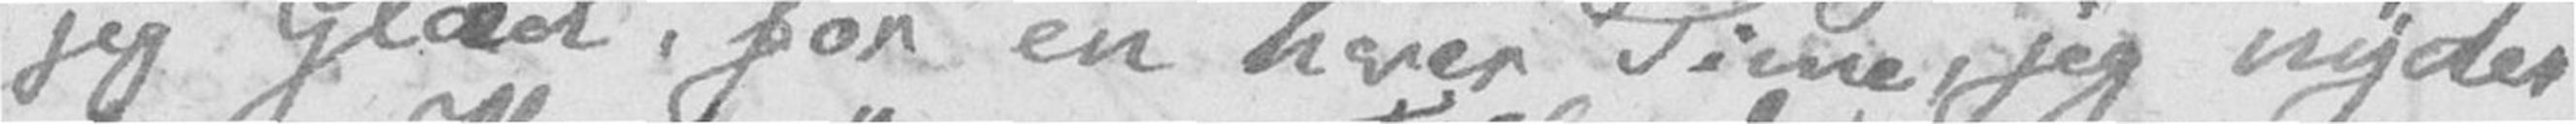jeg Glad, for en hver Time, jeg nyder
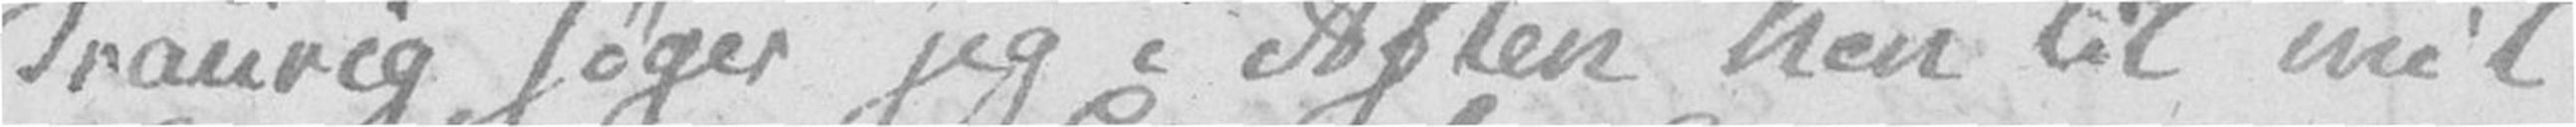Traurig søger jeg i Aften hen til mit
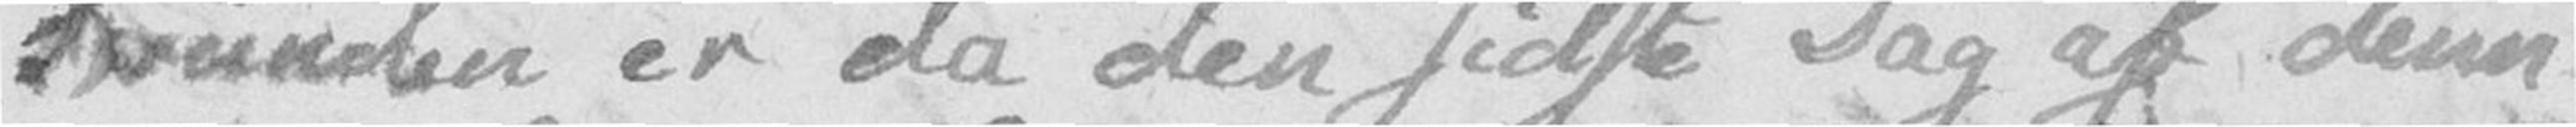Svunden er da den sidste Dag af denn
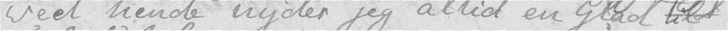ved hende nyder jeg altid en Glad til
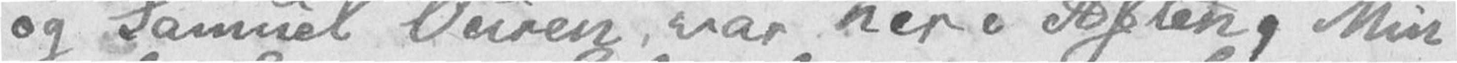og Samuel Ouren, var her i Aften, Min
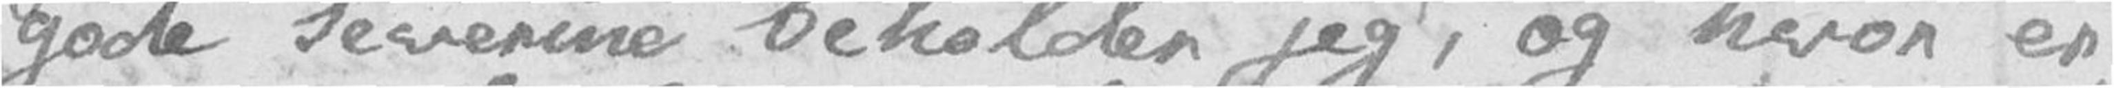gode Severine beholder jeg, og hvor er
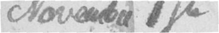November 1ste
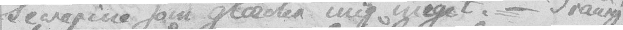Severine som glæder mig meget. – Traurig
